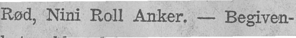Rød, Nini Roll Anker. - Begiven-
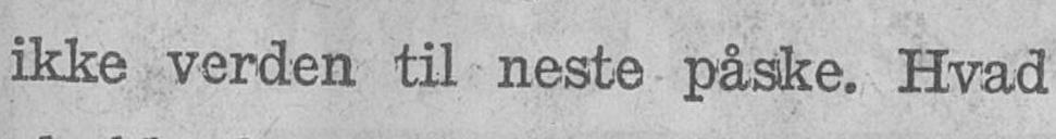ikke verden til neste påske. Hvad
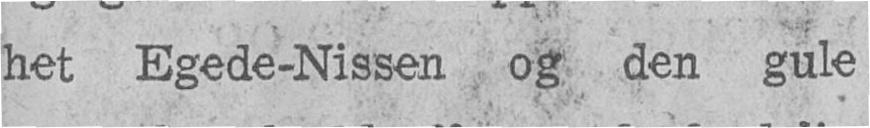het Egede-Nissen og den gule
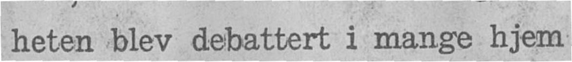heten blev debattert i mange hjem
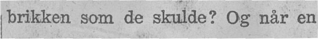brikken som de skulde? Og når en
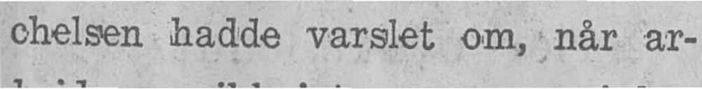chelsen hadde varslet om, når ar-
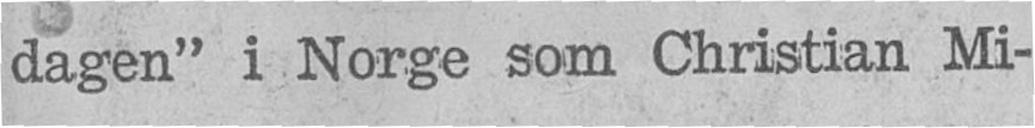dagen" i Norge som Christian Mi-
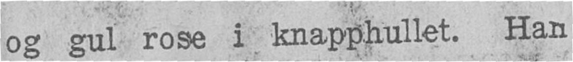og gul rose i knapphullet. Han
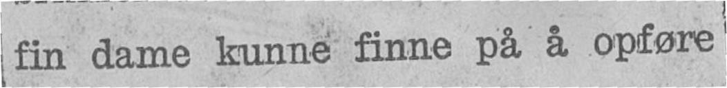fin dame kunne finne på å opføre
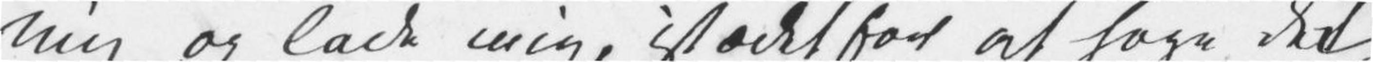mig og lade mig, istædetfor at søge det
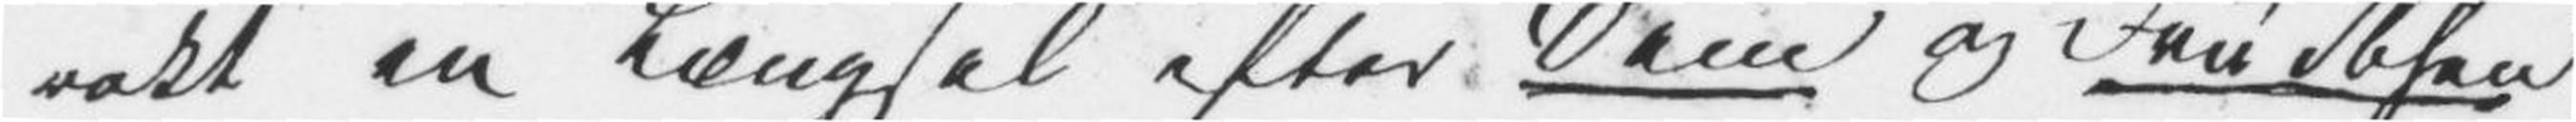vakt en Længsel efter Dem og Fru Ibsen
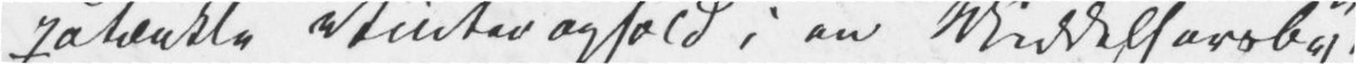påtænkte Vinterophold i en Middelhavsby,
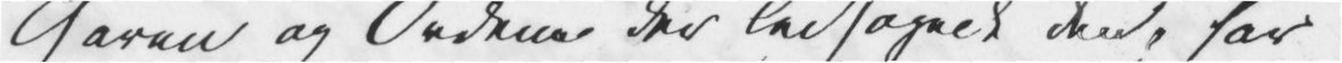Gaven og Ordene der ledsagede den, har
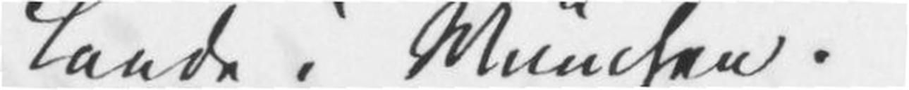lande i München.
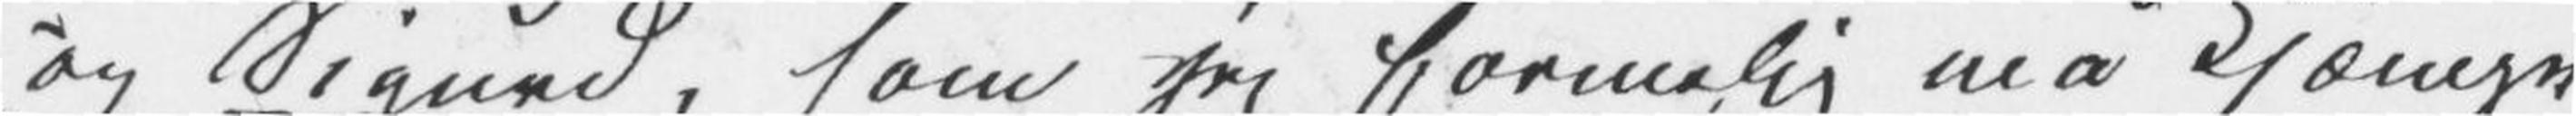og Sigurd, som jeg formelig må kjæmpe
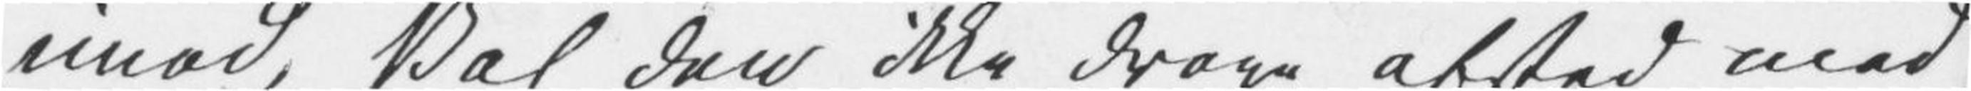imod, skal den ikke drage afsted med
